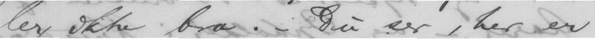ler ikke bra. Du ser, her er
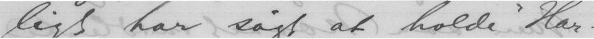ligt har søgt at holde "Har-
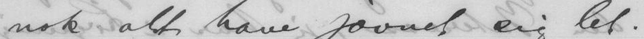nok alt have jævnet sig let.
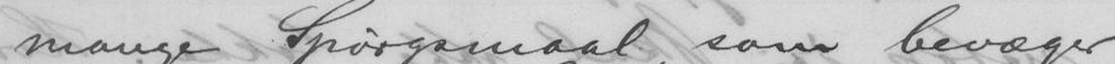mange Spørgsmaal, som bevæger
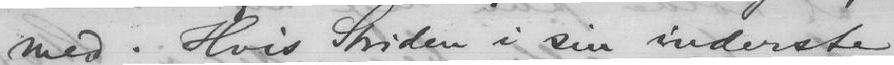med. Hvis Striden i sin inderste
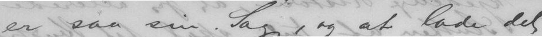er saa sin Sag, og at lade det
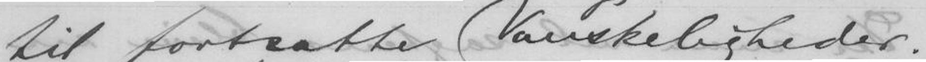til fortsatte Vanskeligheder.
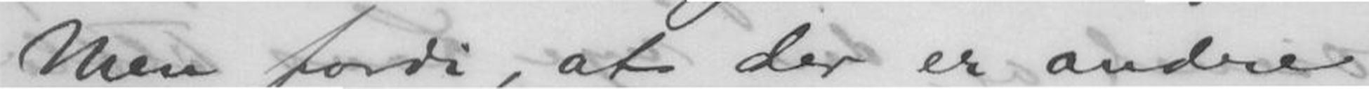Men fordi, at der er andre
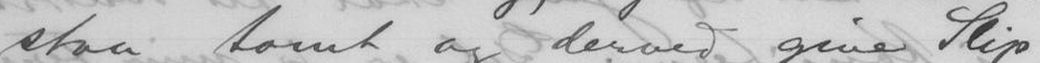staa tomt og derved give Slip
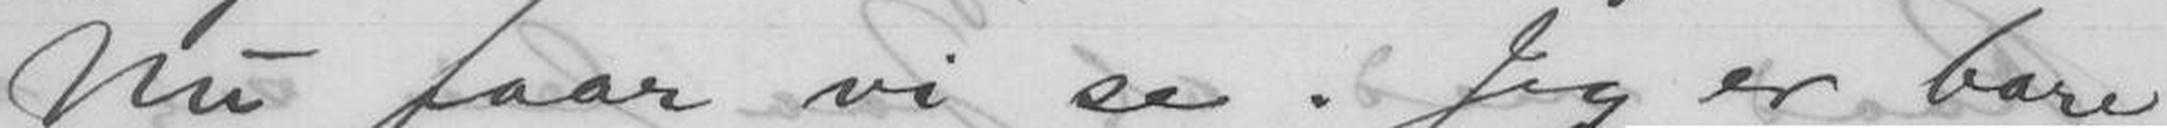Nu faar vi se. Jeg er bare
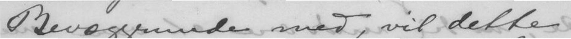Bevæggrunde med, vil dette
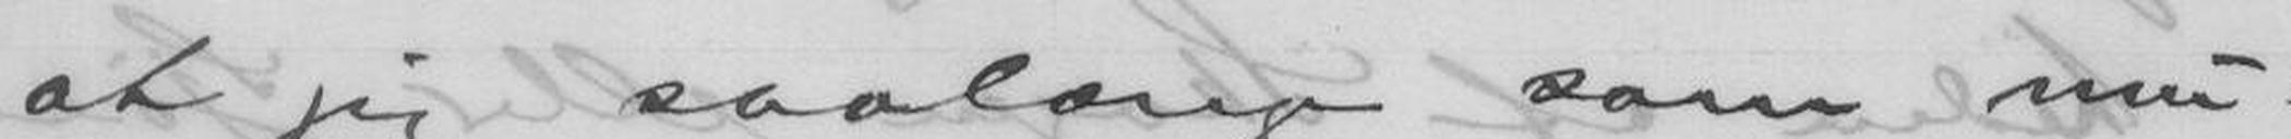at jeg saalænge som mu-
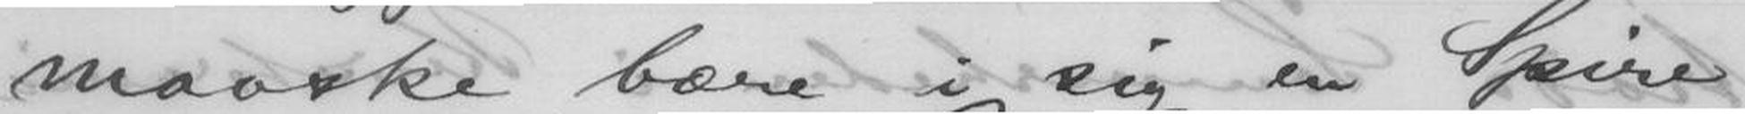maaske bære i sig en Spire
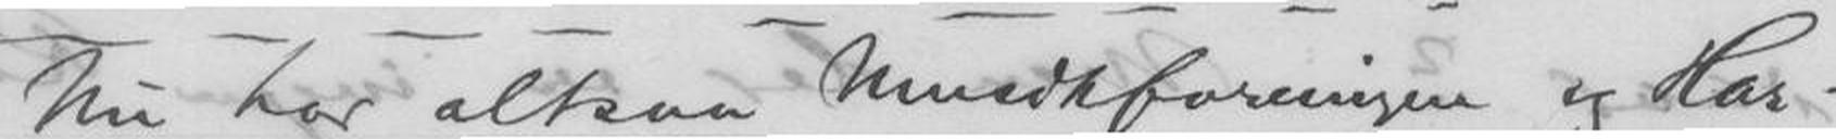Nu har altsaa Musikforeningen og Har-
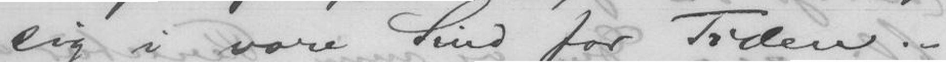sig i vore Sind for Tiden. -
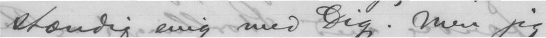stændig enig med Dig. Men jeg
